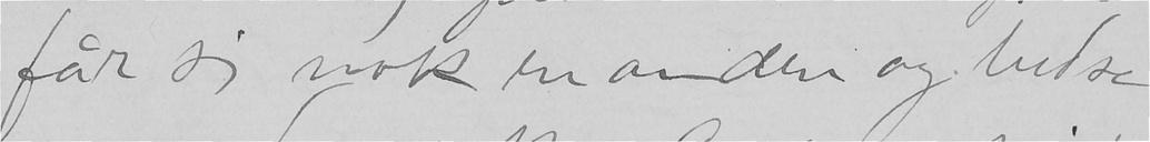får sig nok en anden og bedre
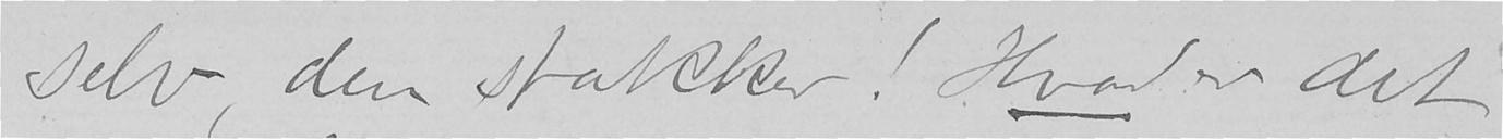selv, den stakker! Hvad er det
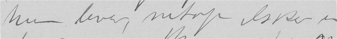hun lever, netop elsker en
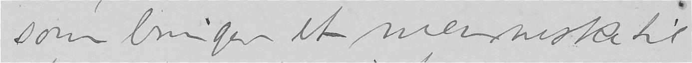som bringer et menneske til
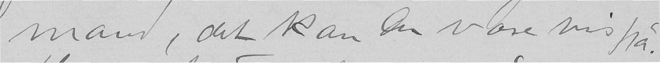mand, det kan Du være vis på.
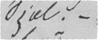Sjæl. -
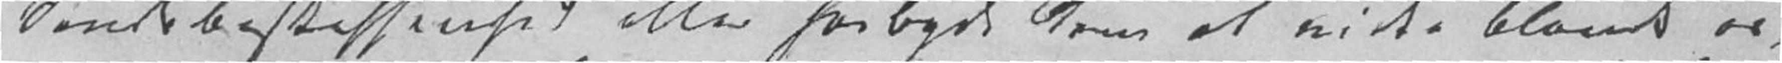Sandsebeskaffenhed eller forbyde dem at virke blandt os,
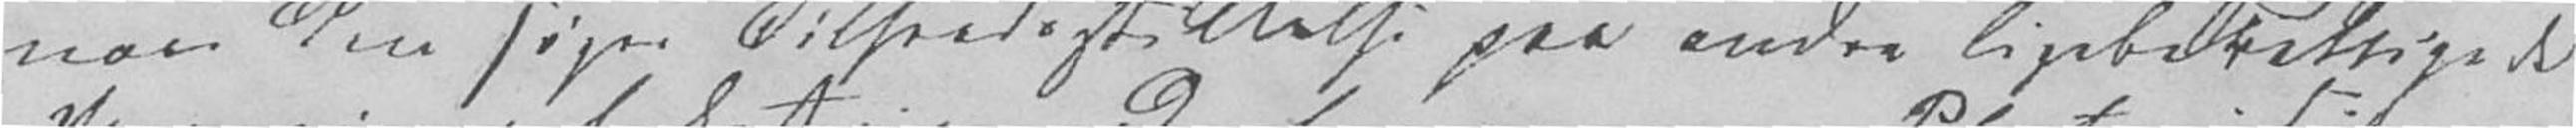naar den søger Tilfredsstillelse paa andre ligeberettigede
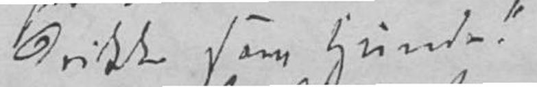drikke som Hunder."
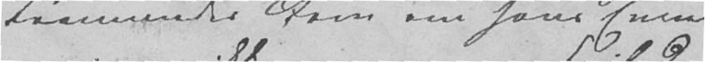Fremmedes Dom om hans Evne
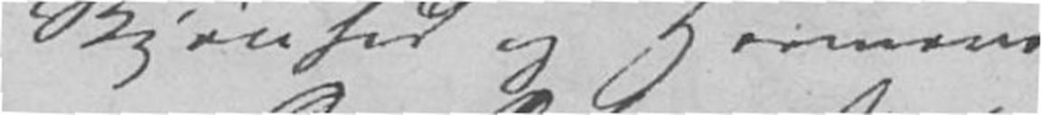Skjønhed og Harmoni
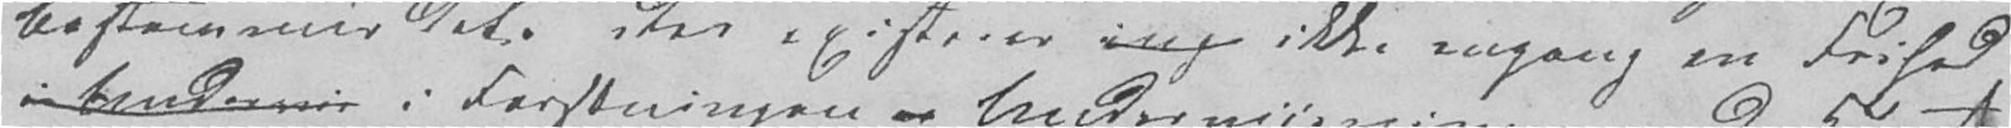bestemmer det. Der existerer ing ikke engang en Frihed
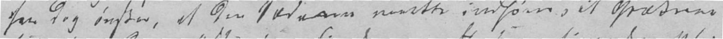han dog ønsker, at den Sædvane maatte indhøver, at Grækerne
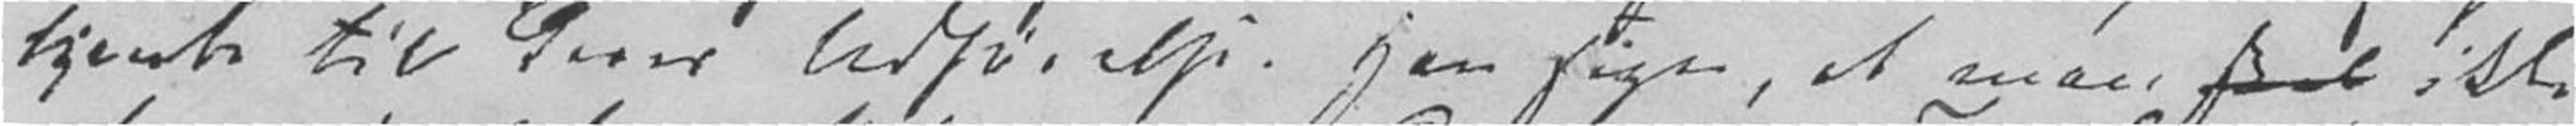tjente til deres Udførelse. Han søger, at man skal ikke
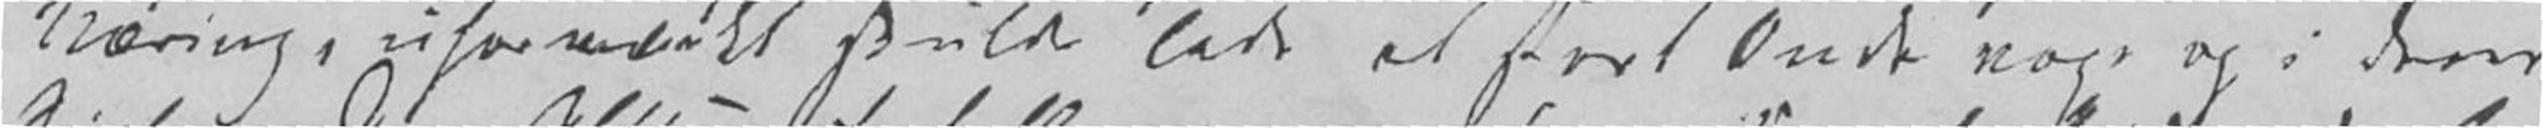Næring, uformærkt skulde lade et stort Onde voxe op i deres
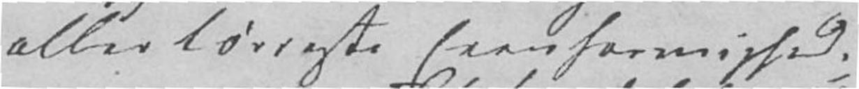aller tørreste Eensformighed.
